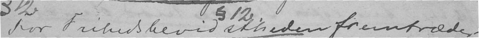For Frihedsbevidstheden fremtræder
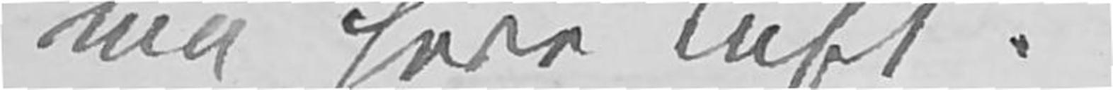må have Luft.
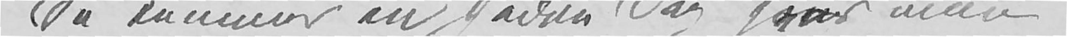Så kommer en sådan Dag hvor man
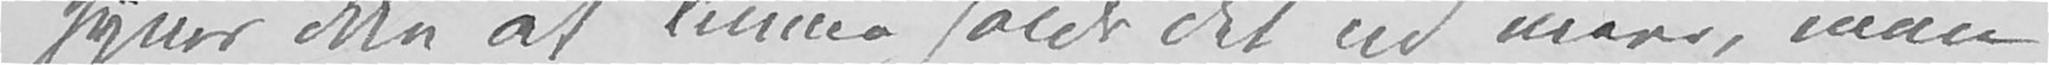synes ikke at kunne holde det ud mere, man
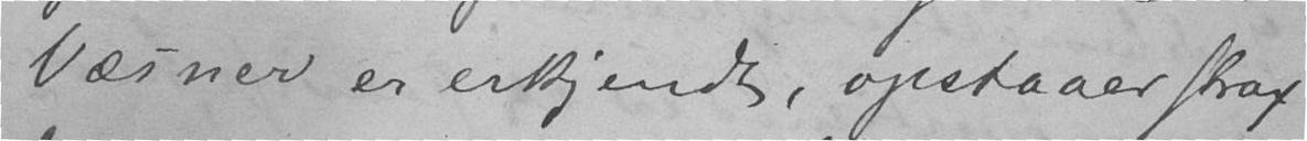Væsner er erkjendt, opstaaer strax
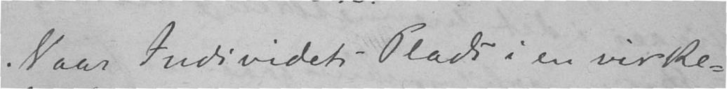Naar Individets Plads i en virke-
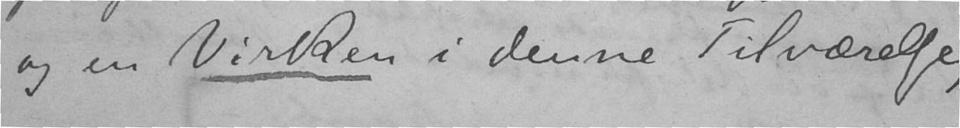og en Virken i denne Tilværelse,
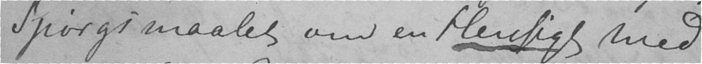Spørgsmaalet om en Hensigt med
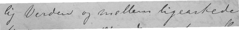lig Verden og mellem ligeartede
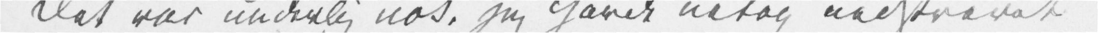Det var underlig nok, jeg havde netop nedskrevet
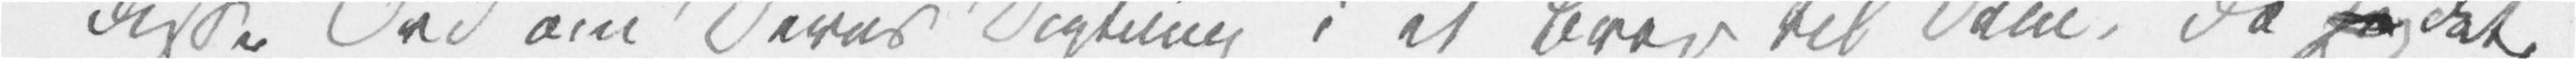disse Ord om Deres Digtning i et Brev til Dem, da jeg det,
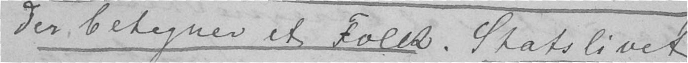der betegner et Folk. Statslivet
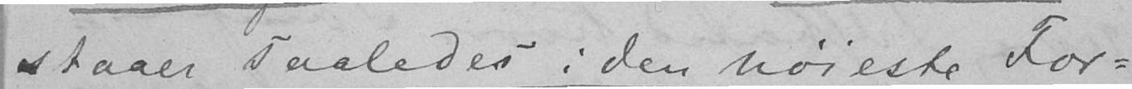staaer saaledes i den høieste For-
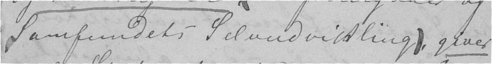Samfundets Selvudvikling), giver
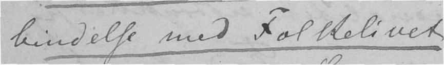bindelse med Folkelivet
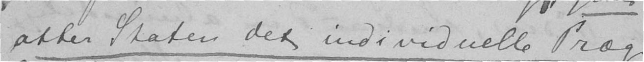atter Staten det individuelle Præg
